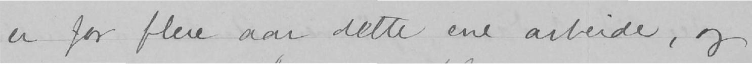er for flere aar dette ene arbeide, og
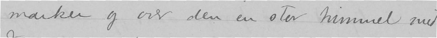marker og over den en stor himmel med
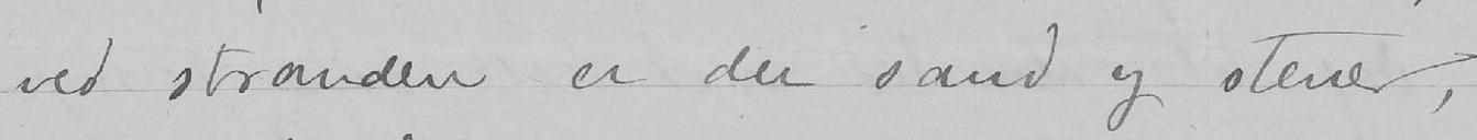ved stranden er der sand og stener,
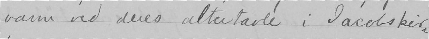varm ved deres altertavle i Jacobskirken,
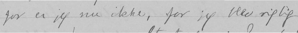for er jeg nu ikke, for jeg blev rigtig
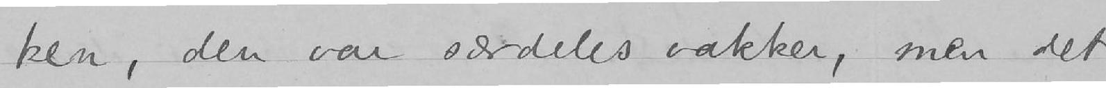den var særdeles vakker, men det
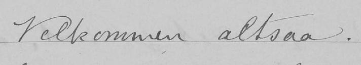Velkommen altsaa.
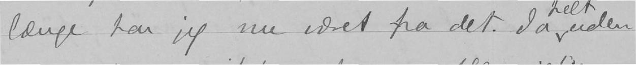længe har jeg nu været fra det. Ja uden-
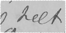helt
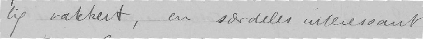lig vakkert, en særdeles interessant
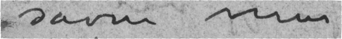savne min
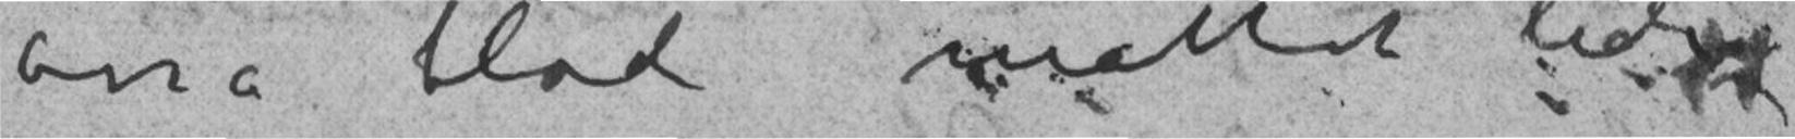osså blot måttet lide
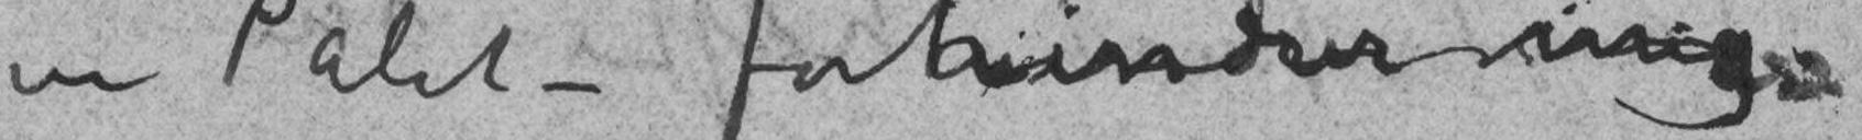en Palet – forhindrer mig
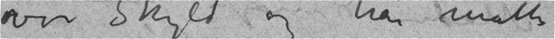vor Skyld og har måttet
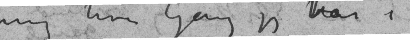mig hver Gang jeg tar i
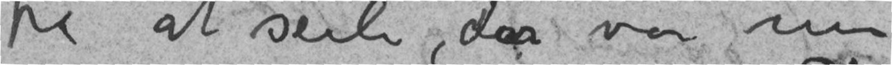fra at seile, der var min
